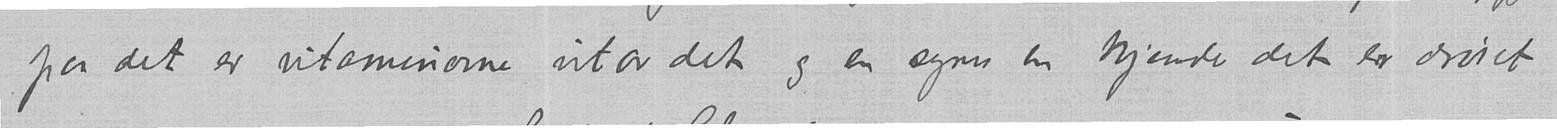paa det er vitaminerne utav det og en synes en kjender det er drøiet
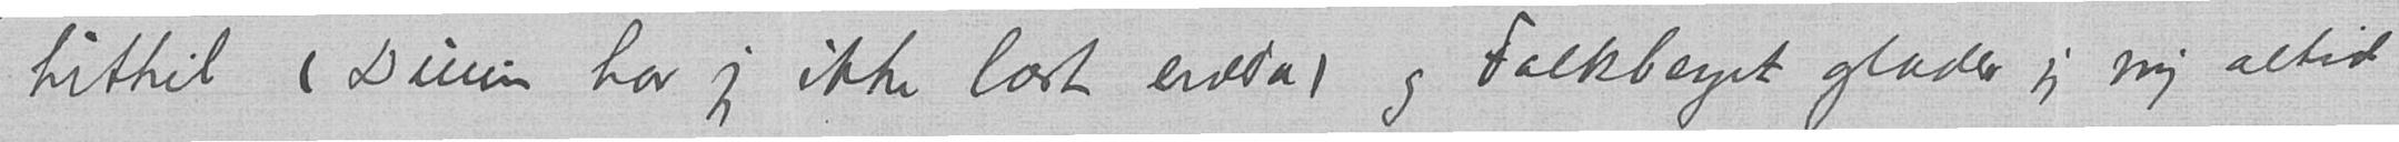hittil (Duun har jeg ikke læst endda) og Falkberget glæder jeg mig altid
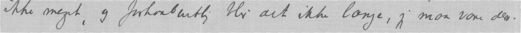ikke meget, og forhaabentlig blir det ikke længe, jeg maa være der.
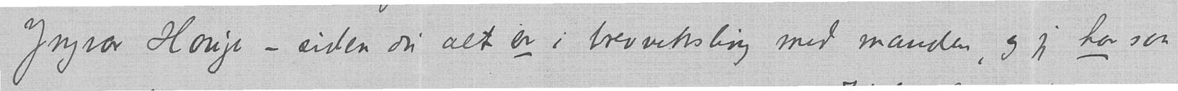Yngvar Hauge – siden du alt er i brevveksling med manden, og jeg har saa
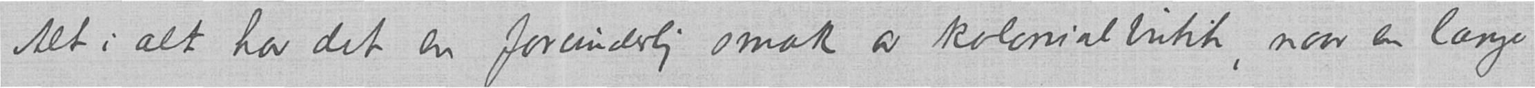Alt i alt har det en forunderlig smak av kolonialbutik, naar en længe
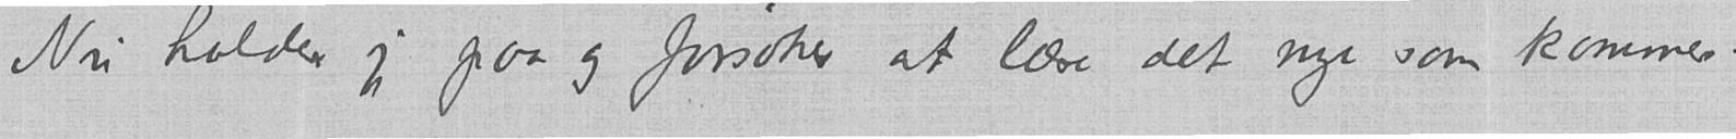Nu holder jeg paa og forsøker at læse det nye som kommer.
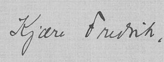Kjære Fredrik,
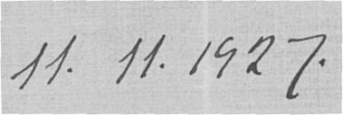11.11.1927.
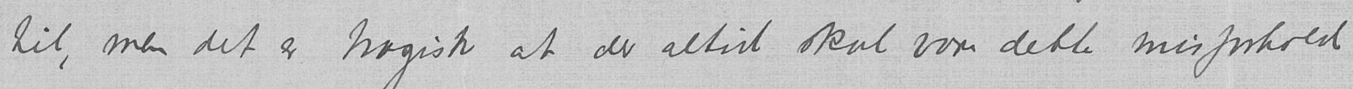til, men det er tragisk at der altid skal være dette misforhold
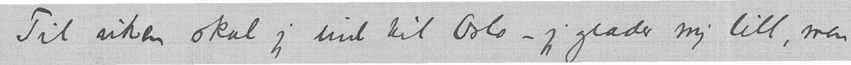Til uken skal jeg ind til Oslo – jeg glæder mig litt, men
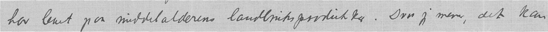har levet paa middelalderens landbruksprodukter. Dvs jeg mener, det kan
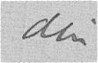din
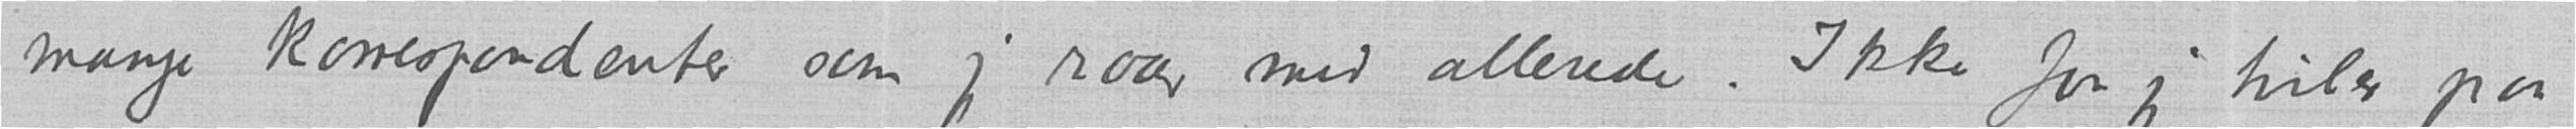mange korrespondenter som jeg raar med allerede. Ikke for jeg tviler paa
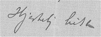Hjertelig hilsen
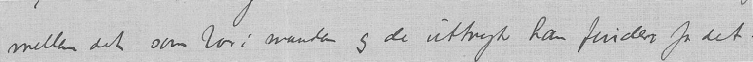mellem det som bor i manden og de uttryk han finder for det.
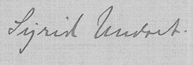Sigrid Undset.
